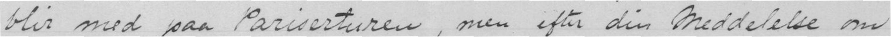blir med paa Pariserturen, men efter din meddelese om
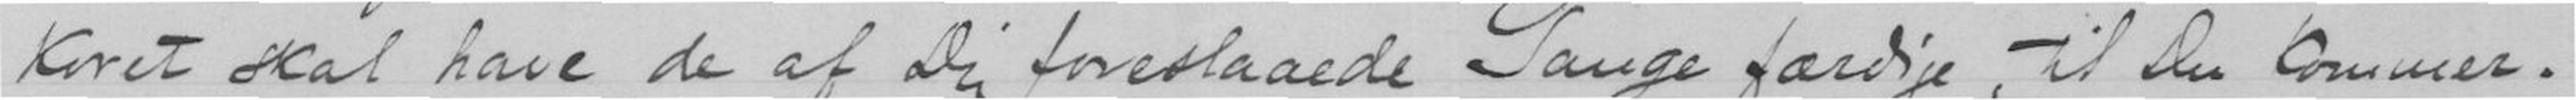Koret skal have de af Dig foreslaaede Sange færsje, til Du kommer.
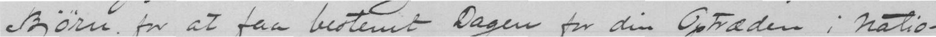Bjørn for at faa bestemt Dagen for din Optræden Natio-
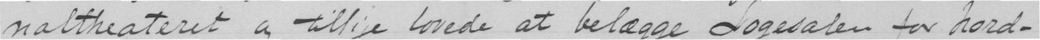naltheateret og tillige lovede at beløgge Logesalen for hord-
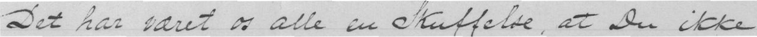Det har vært os alle en Skuffelse, at Du ikke
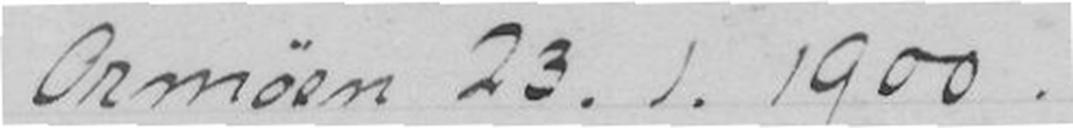Ormøen 23.1.1900
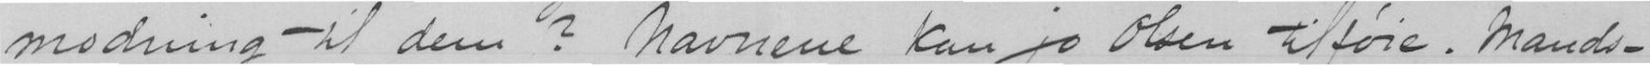modning til dem? Navnene kan jo Olsen tilføie. Mands-
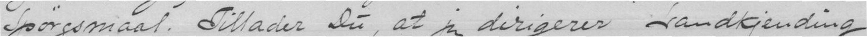Spørgsmaal. Tillader Du, at jeg dirigerer Sandkjending,
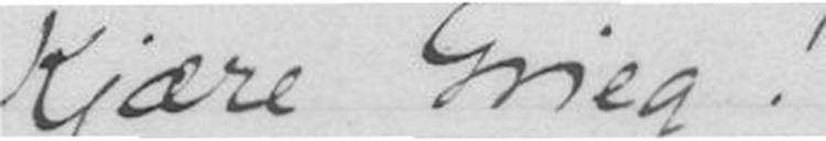Kjære Grieg
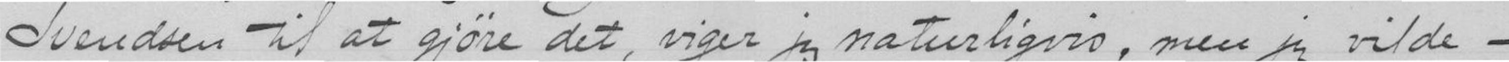Svendsen til at gjøre det,viger jeg naturligvis,men jeg vilde-
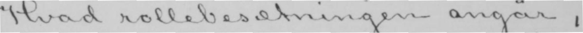Hvad rollebesætningen angår,
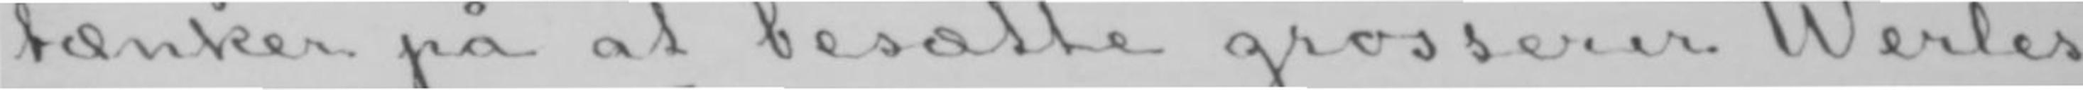tænker på at besætte grosserer Werles
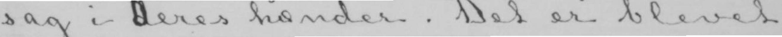sag i dDeres hænder. Det er blevet
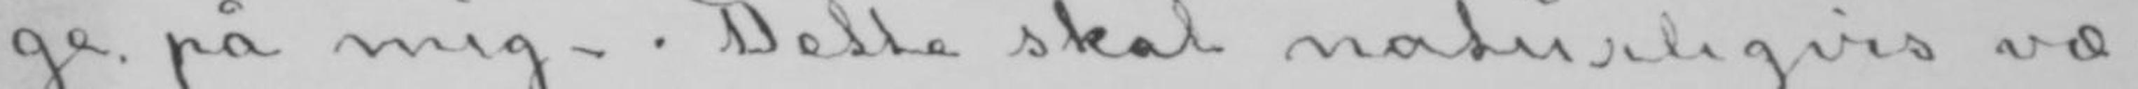ge på mig-. Dette skal naturligvis væ-
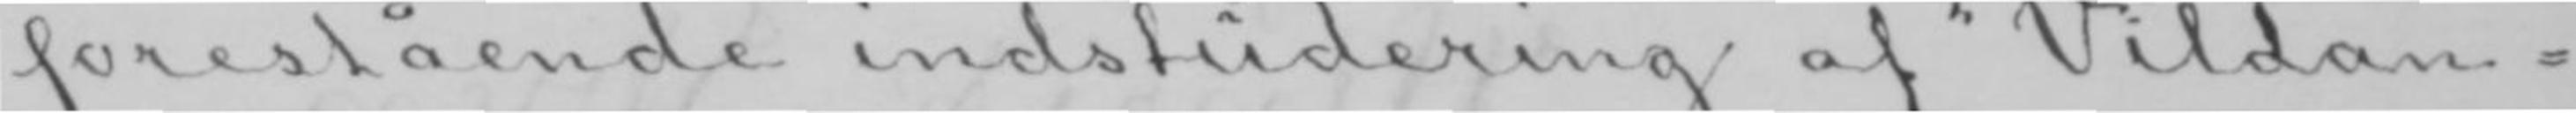forestående indstudering af «Vildan-
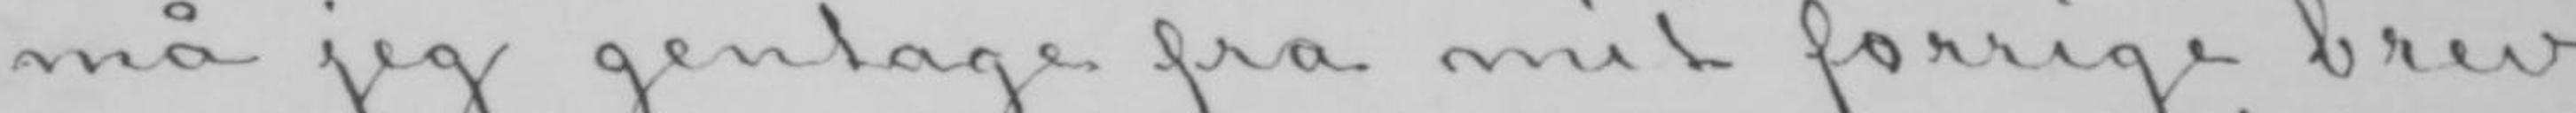må jeg gentage fra mit forrige brev
